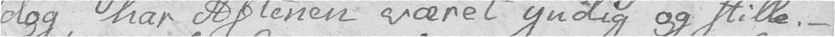dog har Aftenen været yndig og stille. –
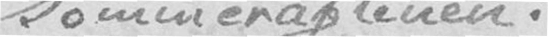Sommeraftenen.
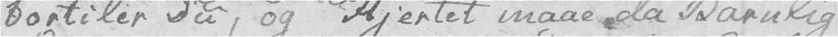bortiler Du, og Hjertet maae da Barnlig
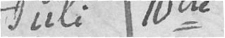Juli 10de
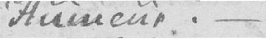Humeur. –
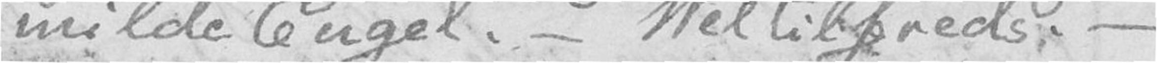milde Engel. – Veltilfreds. –
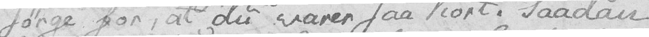sørge for, at du varer saa kort. Saadan
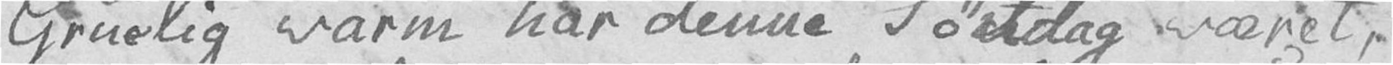Gruelig varm har denne Søndag været,
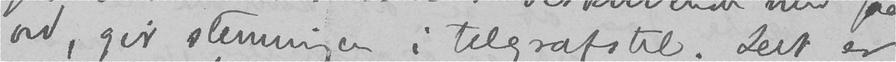ord, gir stemningen i telegrafstil. Det er
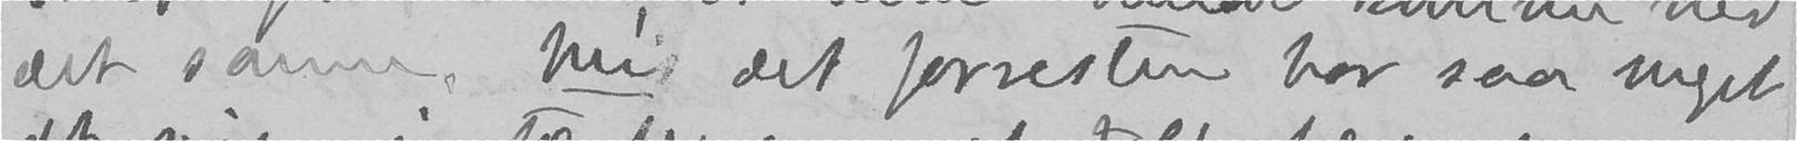det samme, hvis det forresten har saa meget
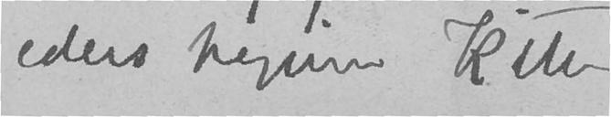eders hengivne Kitty.
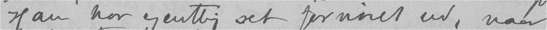Han har egentlig set fornøiet ud, naar
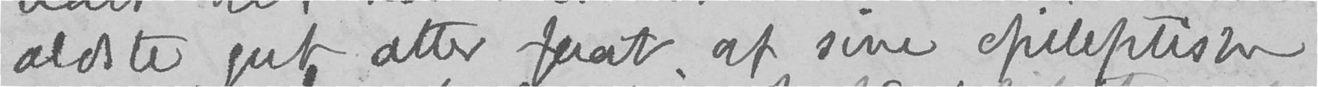ældste gut atter faat af sine epileptiske
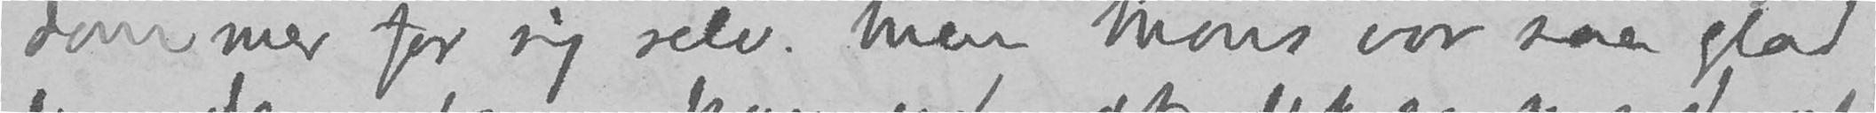dømmer for sig selv. Men Mons var saa glad
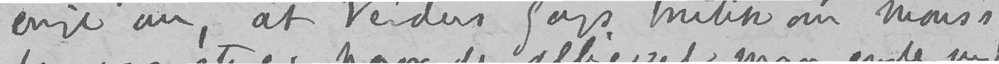enige om, at Verdens Gangs kritik om Monss
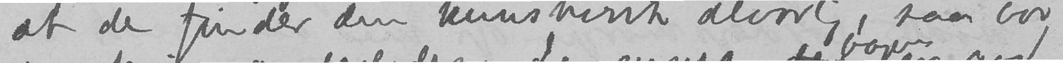at de finder den kunstnerisk alvorlig, saa bør
